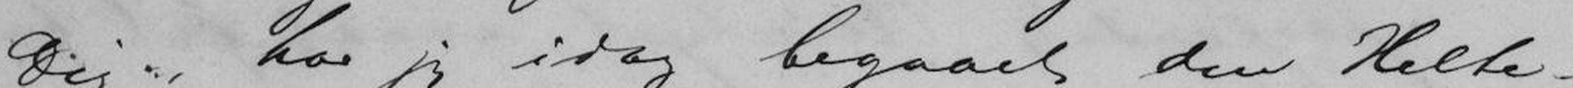Dig, har jeg idag begaaet den Helte-
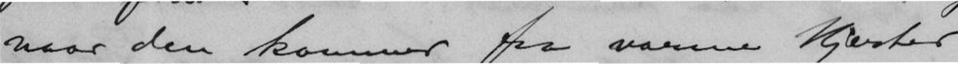naar den kommer fra varme Hjerter
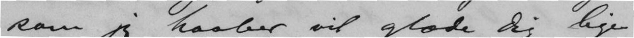som jeg haaber vil glæde dig ligesaa
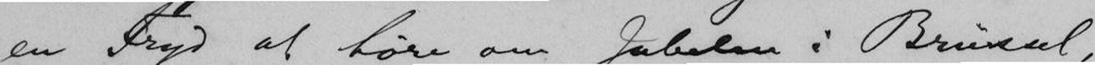en Fryd at høre om Jubelen i Brüssel,
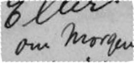ven om.
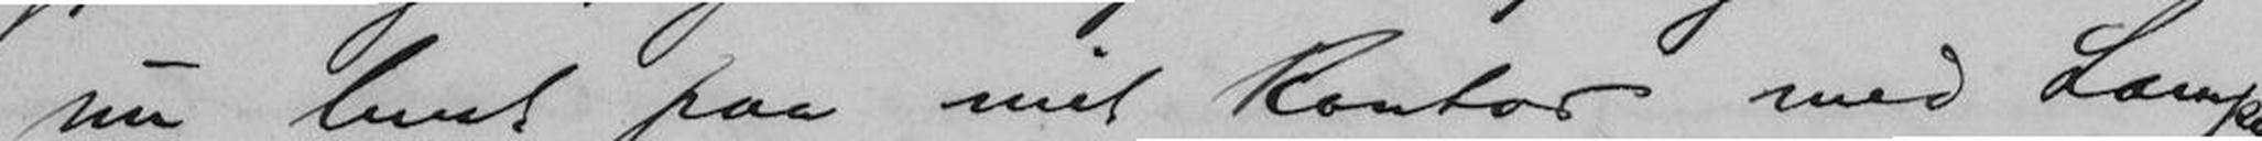nu lunt paa mit Kontor med Lampen.
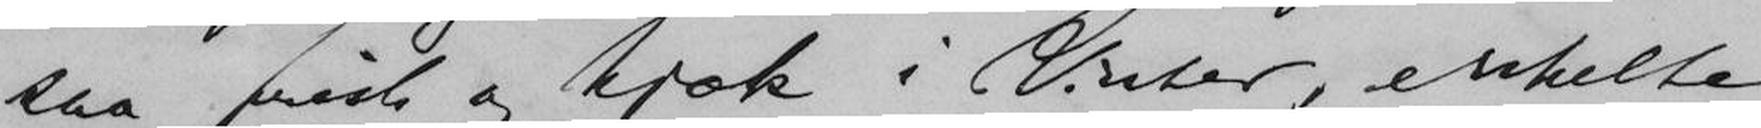saa frisk og kjæk i Vinter, enkelte
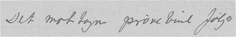Det mottagne prøvebind følger
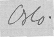Oslo.
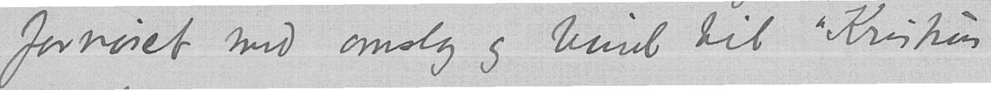fornøiet med omslag og bind til "Kristus
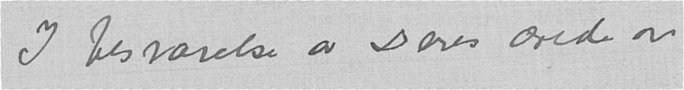I besvarelse av Deres ordre av
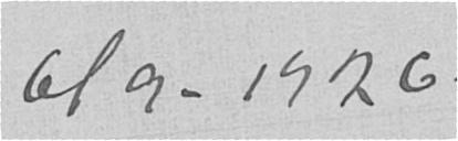6/9-1926.
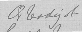Ærbødigst
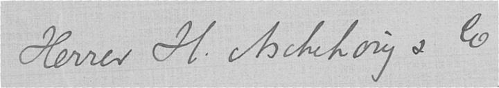Herrer H. Aschehoug & Co
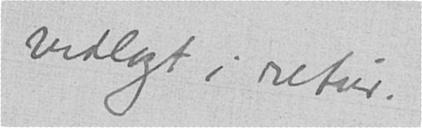vedlagt i retur.
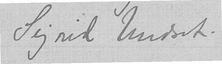Sigrid Undset.
